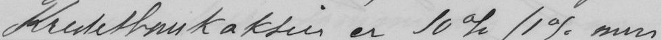Kreditbanken er 10% (1% mere
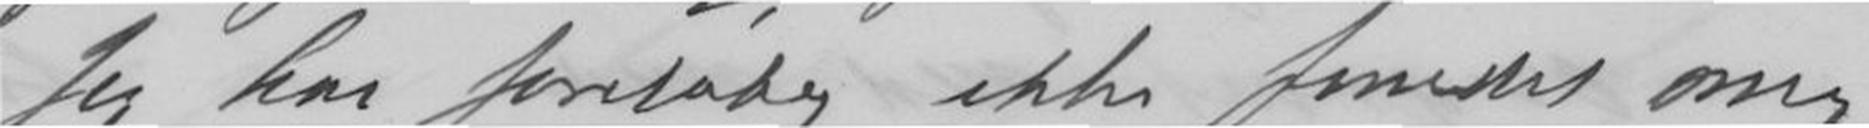Jeg har foreløbig ikke fundet mig
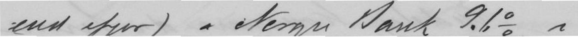end ifjor), i Norges Bank 9.6%, i
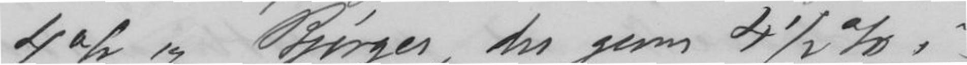4% og Bjørges, det giver 4 1/2% i
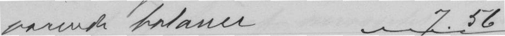varende balance 7.56
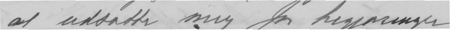at udsætte mig for tilgjøringer
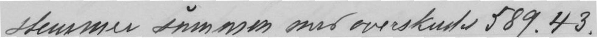stemmer summen over overskudd 589.43.
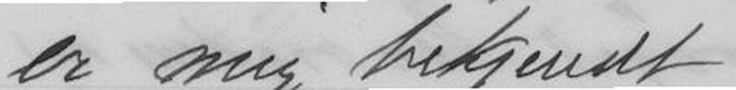er mig bekjendt.
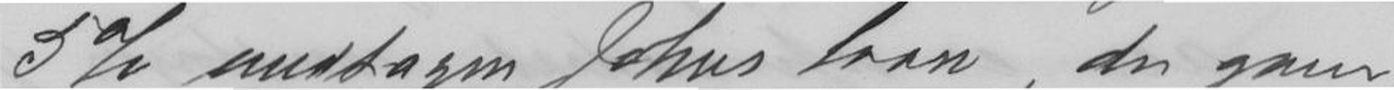5% undtagen Johns laan, det giver
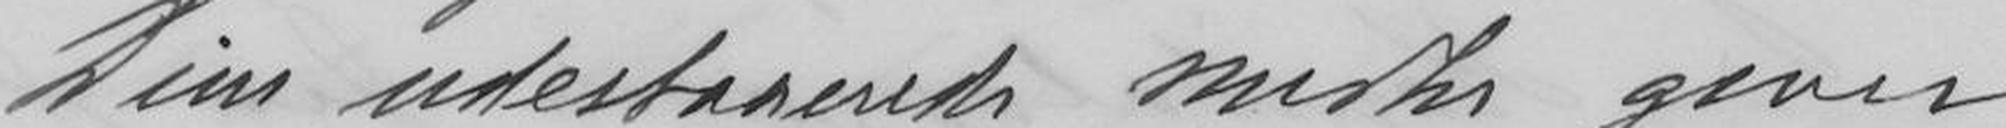Dine udestaaende midler giver
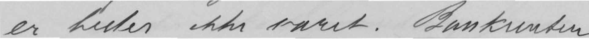er beder ikke været. Bankrenten
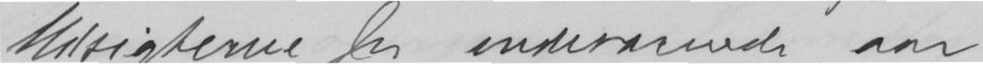Udsigterne for indeværende aar
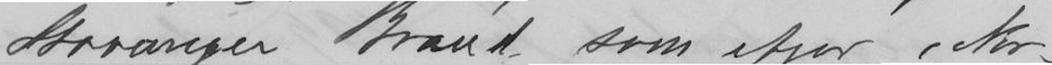Stavanger Brand som ifjord, Nor-
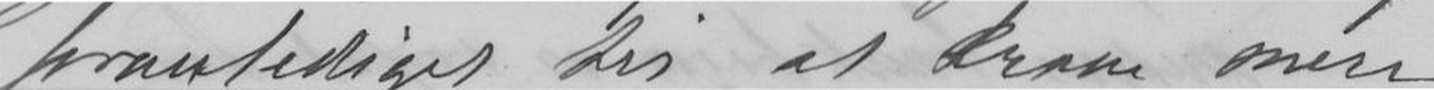foranlediget til at kræve mere
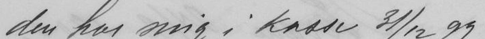den har mig i kasse 31/12 99
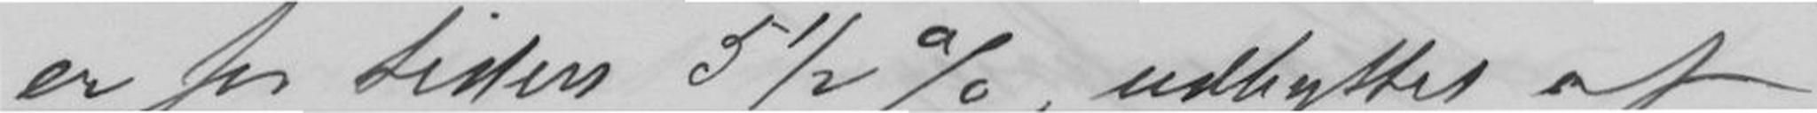er for tiden 5 1/2%, udbyttet af
